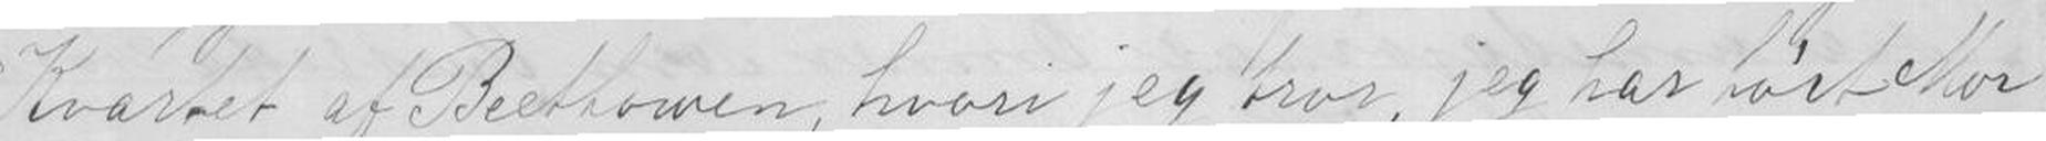Kvartet af Beethowen, hvori jeg tror, jeg har hørt Mor
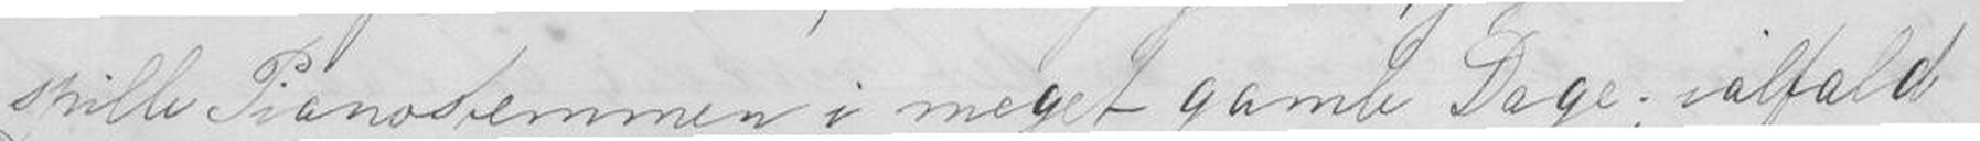spille Pianostemmen i meget gamle Dage; ialfald
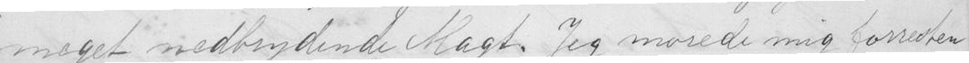meget nedbrydende Magt. Jeg morede mig forresten
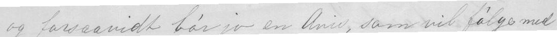og forsaavidt bør jo en Avis, som vil følge med
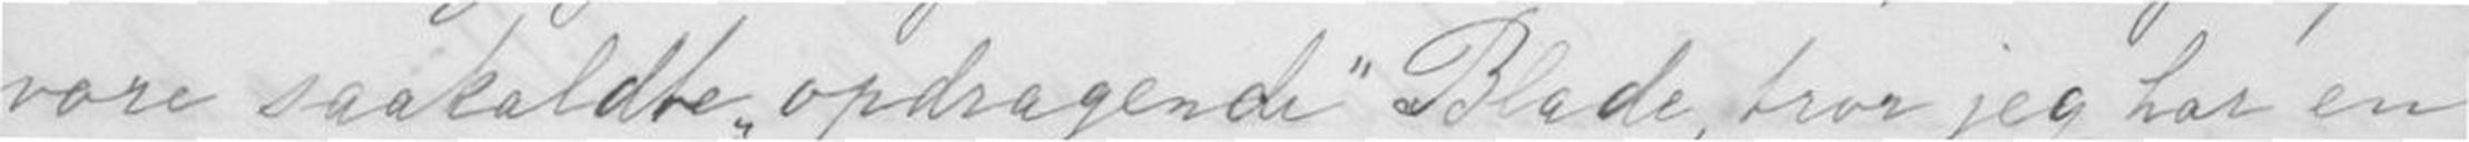vore saakaldte opdragende Blade, tror jeg har en
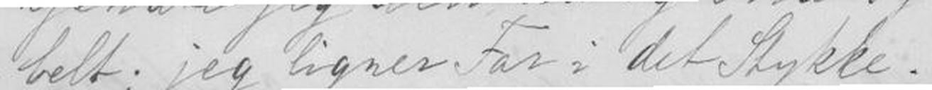belt; jeg ligner Far i det Stykke.
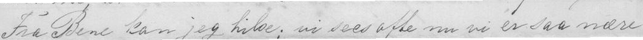Fra Bene kan jeg hilse. vi sees ofte nu vi er saa nære
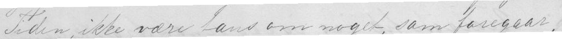Tiden, ikke være taus om noget, som foregaar,
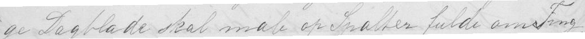ge Dagblade skal male op Spalter fulde om Ting,
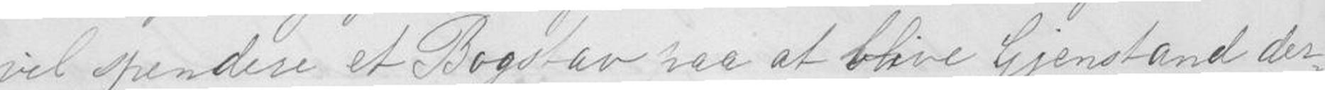vil spendere et Bogstav paa at blive Gjenstand der-
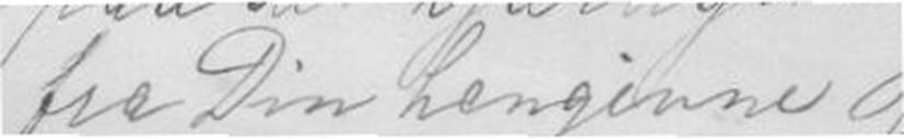fra Din hengivne
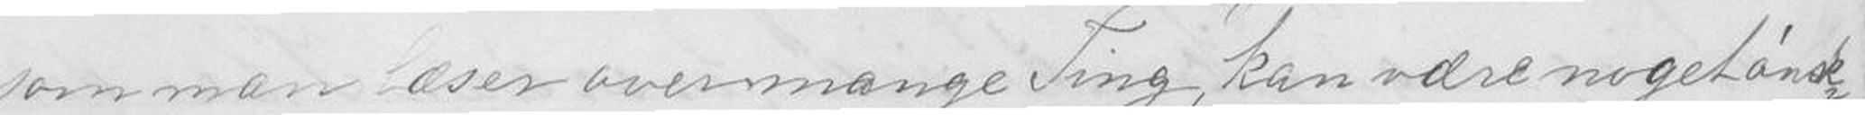som man læser over mange Ting, kan være noget ønsk-
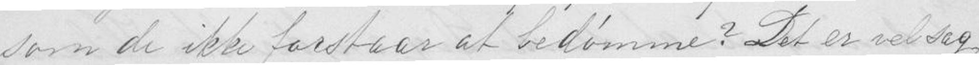som de ikke forstaar at bedømme? Det er vel sag-
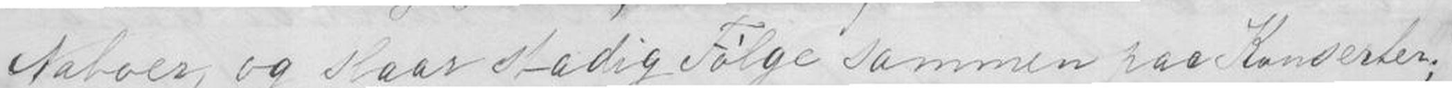Naboer, og slaar stadig Følge sammen paa Konserter;
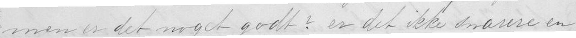men er det noget godt? er det ikke snarere en
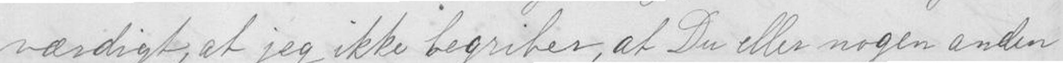værdigt, at jeg ikke begriber, at Du eller nogen anden
